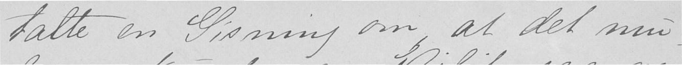talte en Gisning om at det mu-
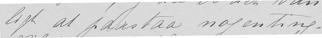ligt at paastaa nogenting.
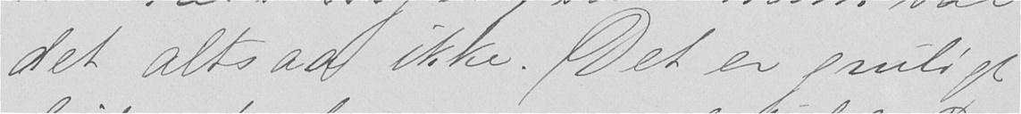det altsaa ikke. Det er gruligt
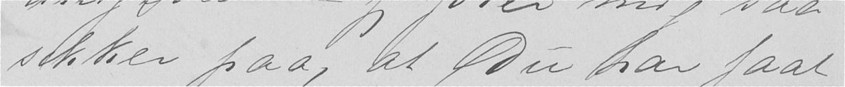sikker paa, at Du har faat
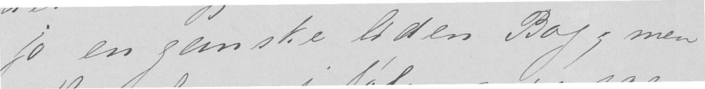jo en ganske liden Bog; men
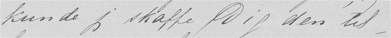kunde jeg skaffe Dig den til-
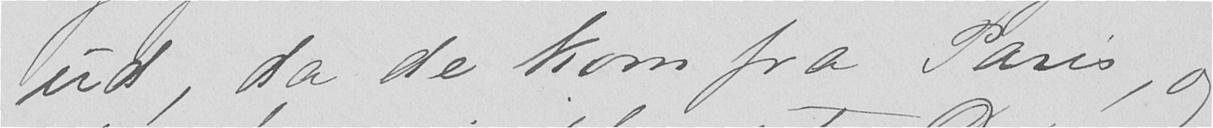ud, da de kom fra Paris, og
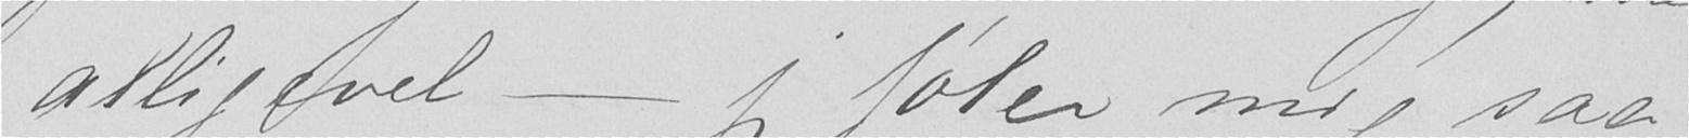alligevel - jeg føler mig saa
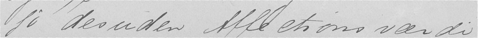jo desuden Affectionsværdi
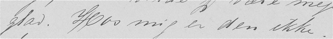glad. Hos mig er den ikke.
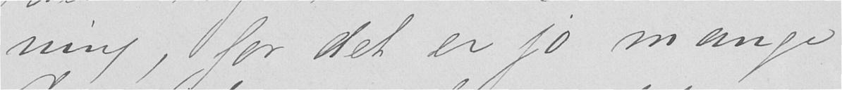ning, for det er jo mange
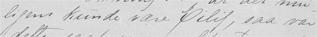ligens kunde være Eilif, saa var
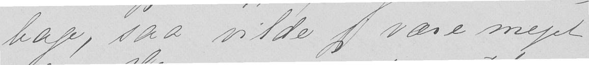bage, saa vilde jeg være meget
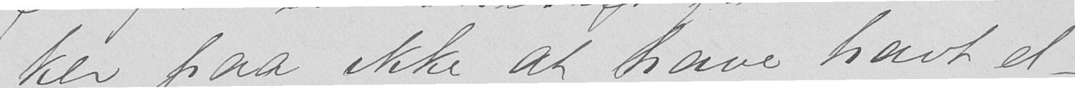ker paa ikke at have havt el-
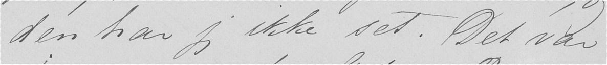den har jeg ikke set. Det var
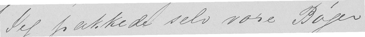Jeg pakkede selv vore Bøger
